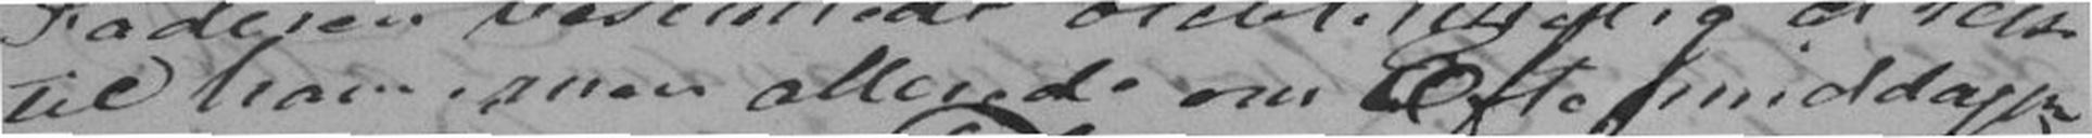til ham, men allerede om Eftermiddagen
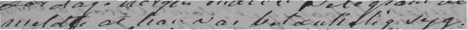meldte at han var betænkelig syg.
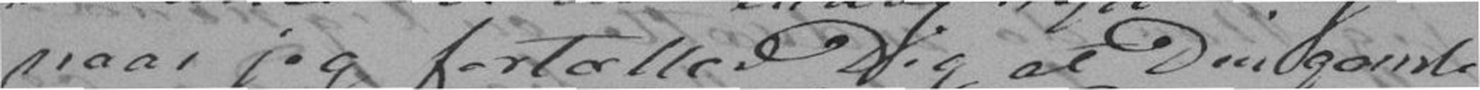naar jeg fortæller Dig at Din gamle
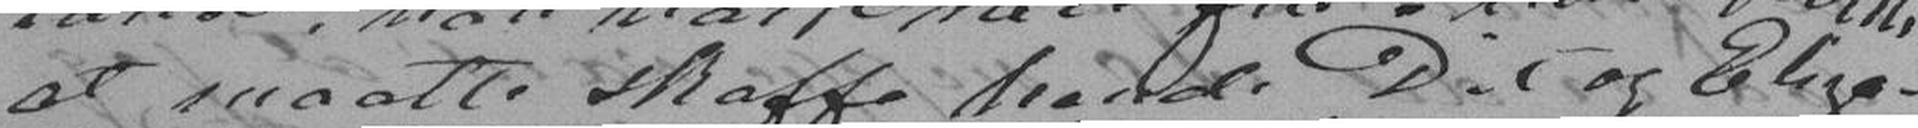at maatte skaffe hende Dit og Eliza-
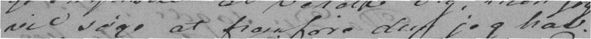vil søge at fremføre dem jeg har.
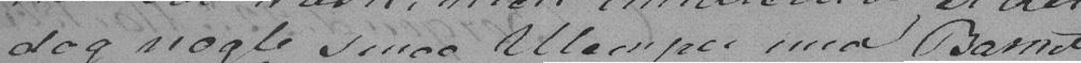dog nogle smaa Ulemper med Barnet,
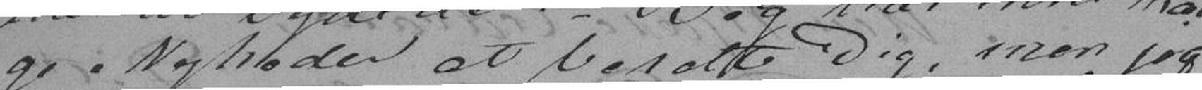ge Nyheder at berette Dig, men jeg
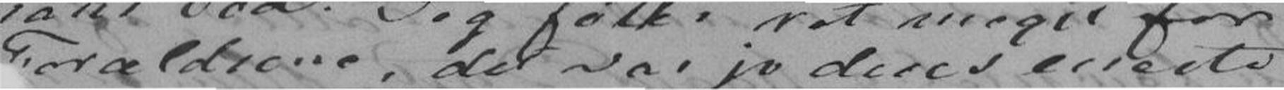Forældrene, det var jo deres eneste
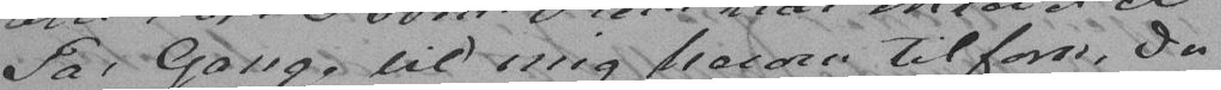Par Gange til mig herom tilforn, Du
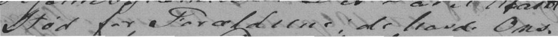Stød for Forældrene; de havde Ons-
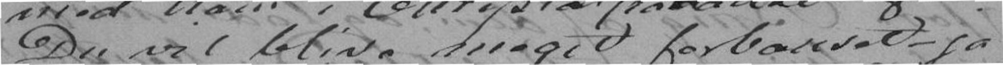Du vil blive meget forbauset - ja
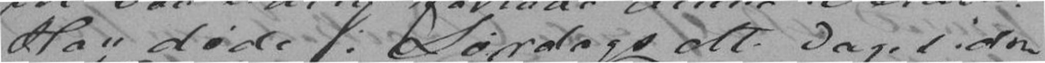Han døde i Lørdags otte Dage siden
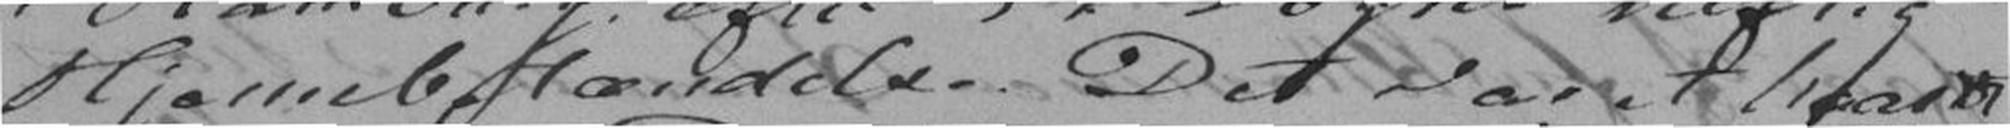Hjernebetændelse. Det var et haart
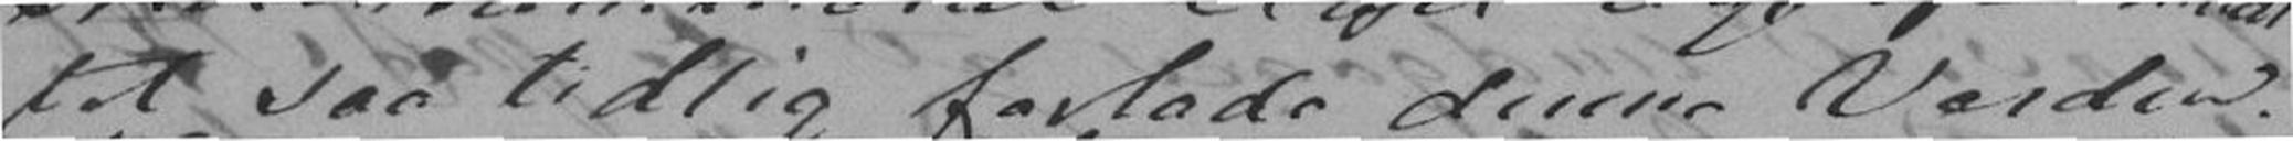tet saa tidlig forlade denne Værden.
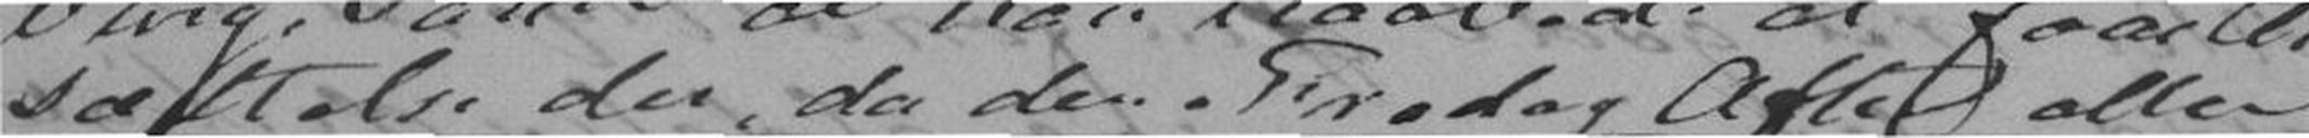sættelse der, da der Fredag Aften eller
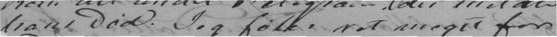hans Død. Jeg føler ret meget for
